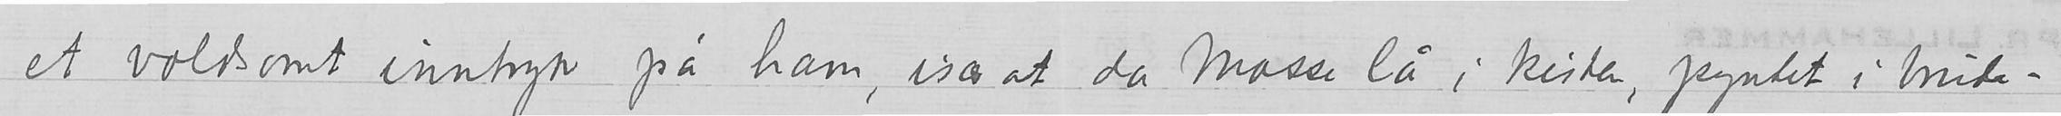et voldsomt inntryk pa ham, især at da Mosse lå i kisten, pyntet i brude –
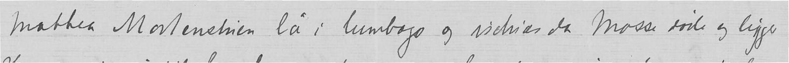Mathea Mortenstuen lå i lumbago og ischiæs da Mosse døde og ligger
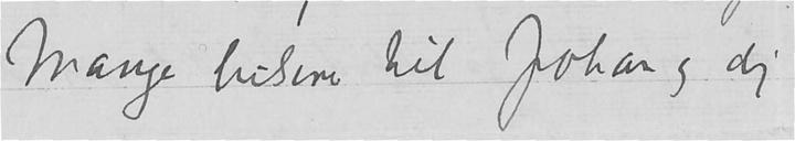Mange hilsener til Johan og dig
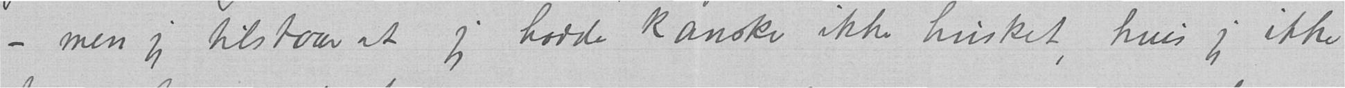- men jeg tilstaar at jeg hadde kanske ikke husket, hvis jeg ikke
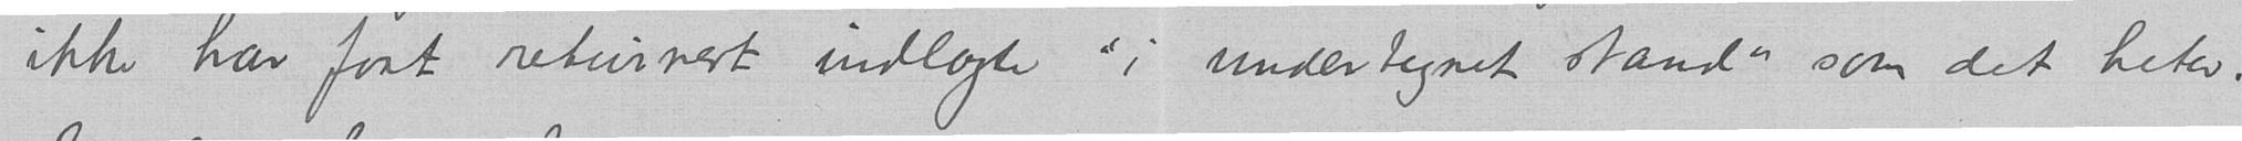ikke har faat returnert indlagte "i undertegnet stand" som det heter.
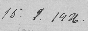15.1.1926.
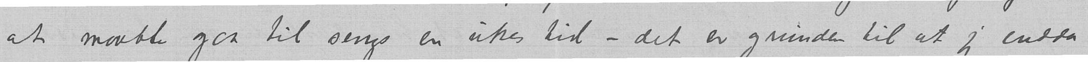at maatte gaa til sengs en ukes tid – det er grunden til at jeg endda
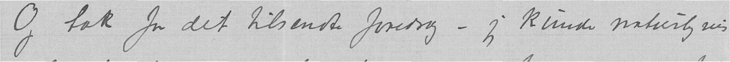Og tak for det tilsendte foredrag – jeg kunde naturligvis
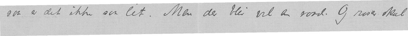saa er det ikke saa let. Men der blir vel en raad. Og roser skal
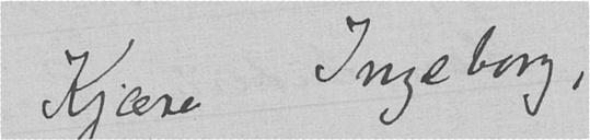Kjære Ingeborg,
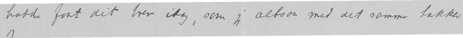hadde faat dit brev idag, som jeg altsaa med det samme takker
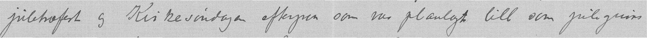juletræfest og Kirkesøndagen efterpaa som var planlagt litt som pilegrims-
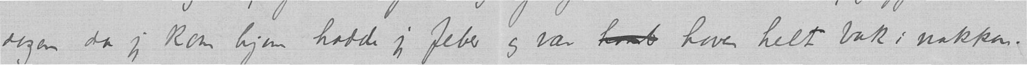dagen da jeg kom hjem hadde jeg feber og var hent hoven helt bak i nakken.
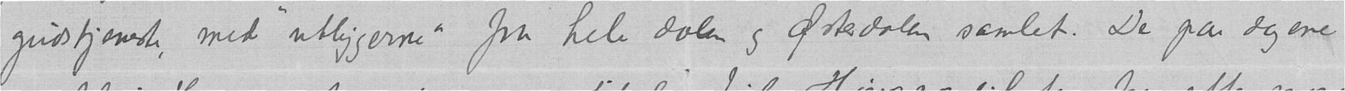gudstjeneste, med "utliggerne" fra hele dalen og Østerdalen samlet. De par dagene
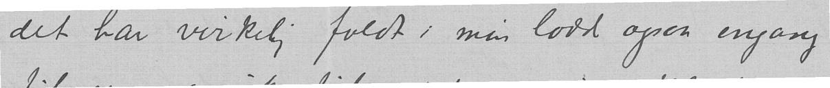det har virkelig faldt i min lodd ogsaa engang
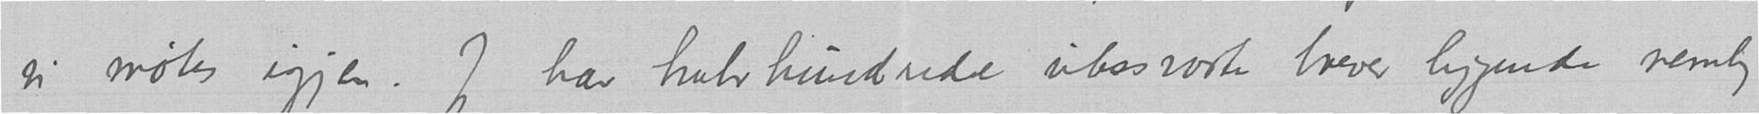vi møtes igjen. Jeg har halvhundrede ubesvarte brever liggende nemlig
